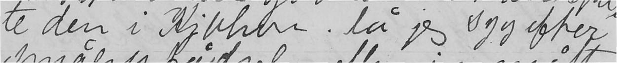te den i Kjbhvn. lå jeg syg efter
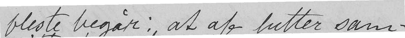fleste begår: at af lutter sam-
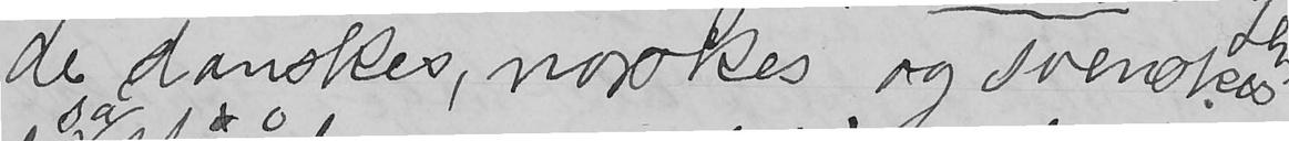de danskes, norskes, og svenskes
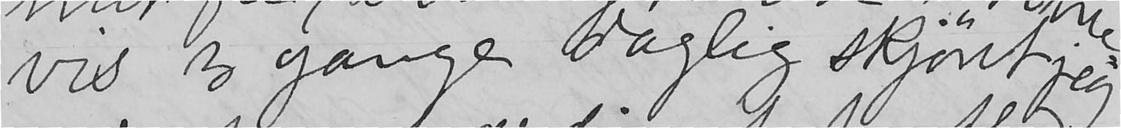vis 3 gange daglig skjønt jeg
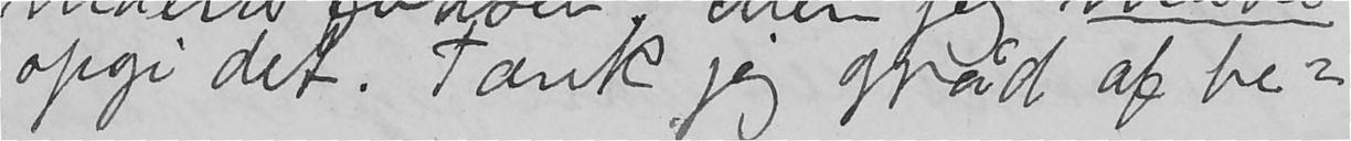opgi det. Tænk jeg gråd af be-
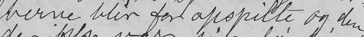verne blir for opspilte og den
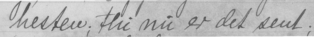hesten; thi nu er det sent;
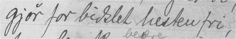gjør for bidslet hesten fri,
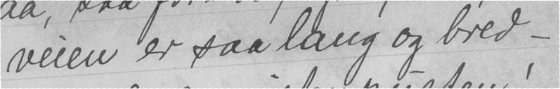veien er saa lang og bred -
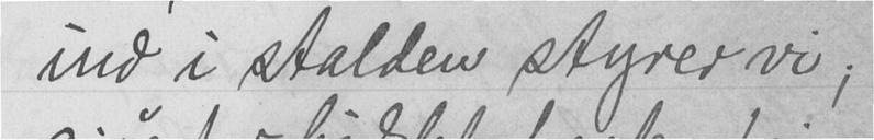ind i stalden styrer vi;
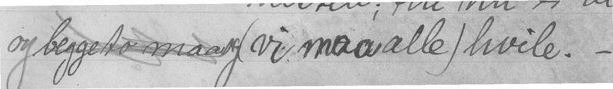og begge to maa og (vi maa alle) hvile. -
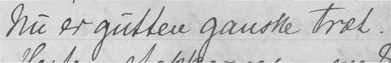nu er gutten ganske træt.
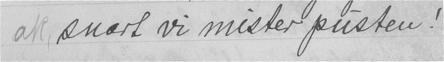ak, snart vi mister pusten!
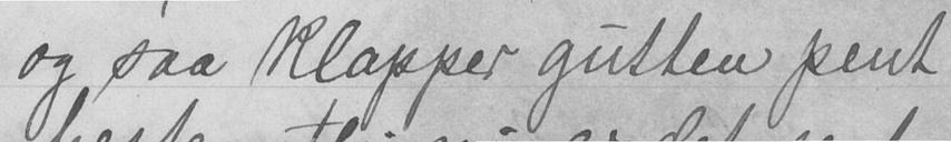og saa klapper gutten pent
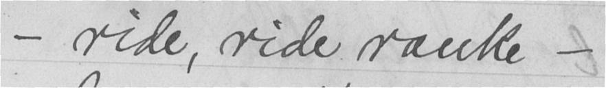- ride, ride ranke -
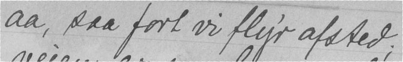aa, saa fort vi fly'r afsted;
